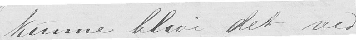kunne blive det ved,
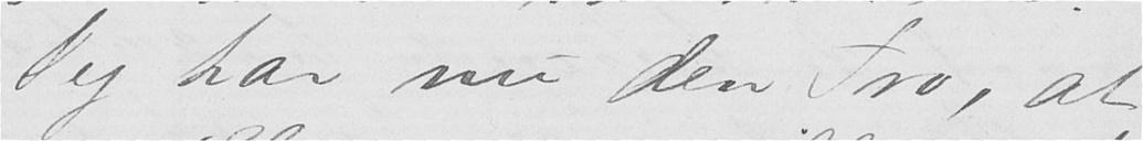Jeg har nu den Tro, at
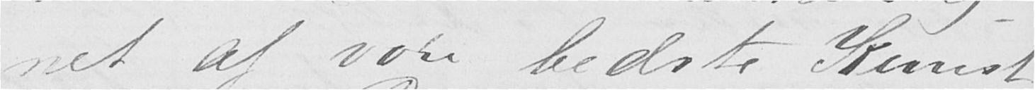net af vore bedste Kunst-
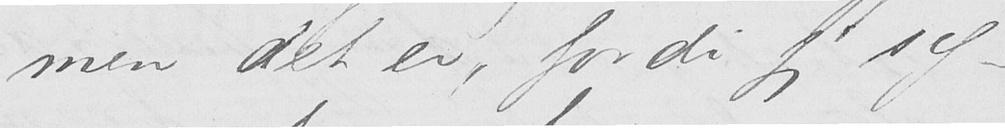men det er, fordi jeg sy-
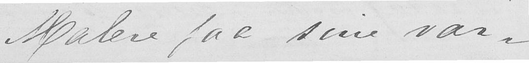Malere faa sine var-
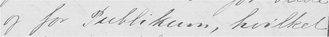og for Publikum, hvilket
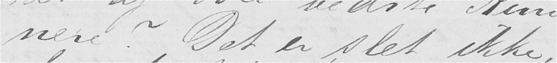nere? Det er slet ikke,
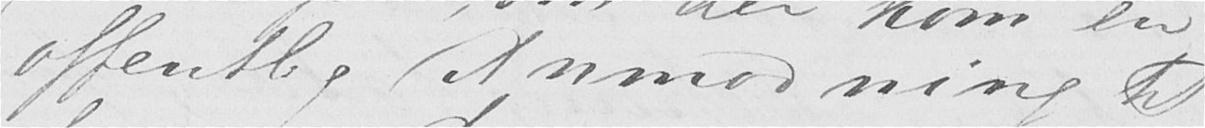offentlig Anmodning til
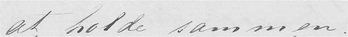at holde sammen.
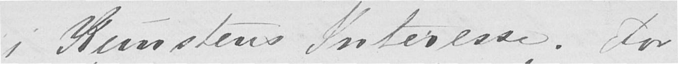i Kunstens Interesse. For
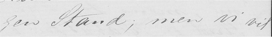gen Stand; men vi vil
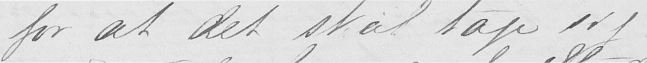for at det skal tage sig
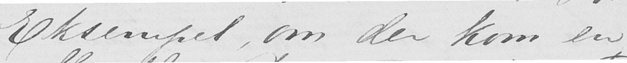Eksempel, om der kom en
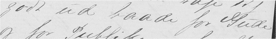godt ud baade for Gude
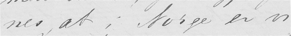nes, at i Norge er vi
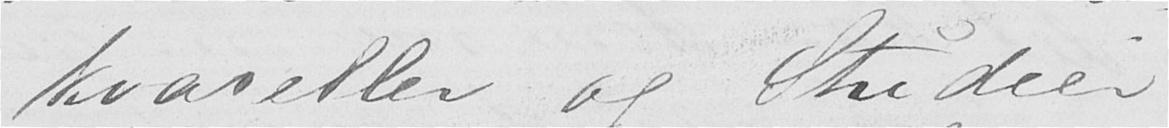kvareller og Studier,
